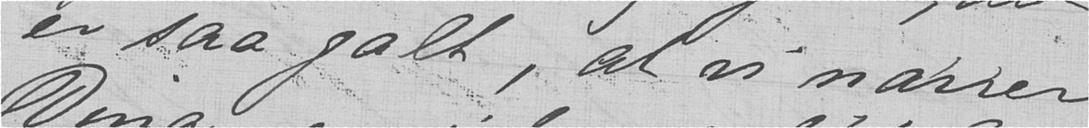er saa galt, at vi narrer
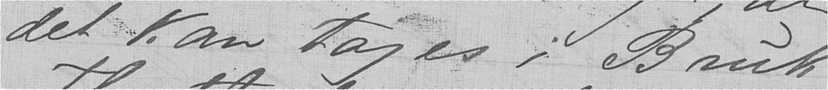det kan tages i Bruk
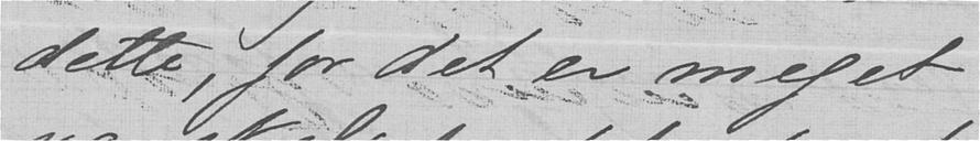dette, for det er meget
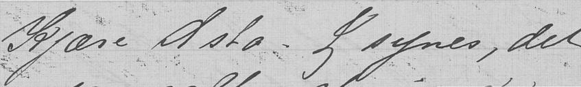Kjære Asta. Jeg synes, det
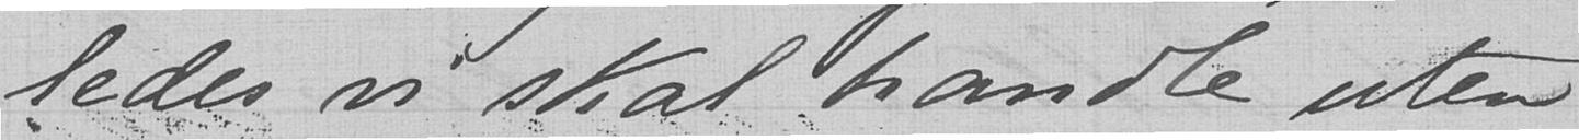ledes vi skal handle uten
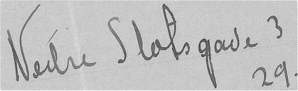Nedre Slotsgade 3
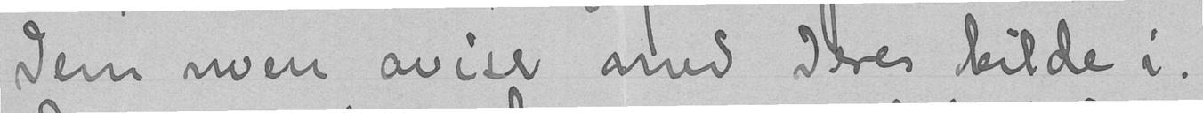Dem noen aviser med Deres bilde i.
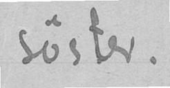søster.
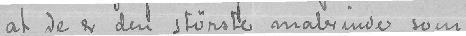at de er den største malerinde som
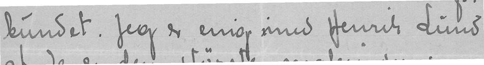bundet. Jeg er enig med Henrik Lund
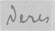Deres
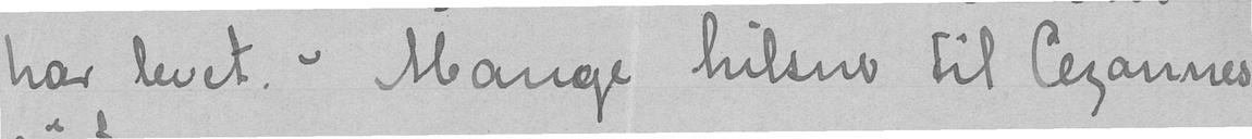har levet. - Mange hilsen til Cezannes
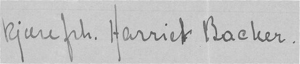Kjære frk. Harriet Backer.
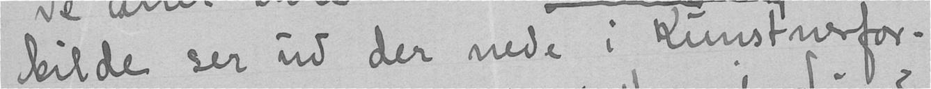bilde ser ud der nede i Kunstnerfor-
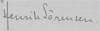Henrik Sørensen.
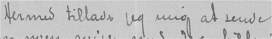Hermed tillader jeg mig at sende
